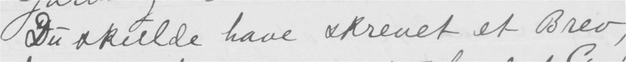Du skulde have skrevet et Brev,
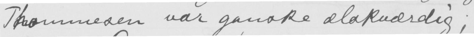Thommesen var ganske elskværdig;
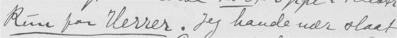Kun for Herrer. Jeg havde nær slaat
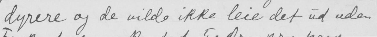dyrere og de vilde ikke leie det ud uden
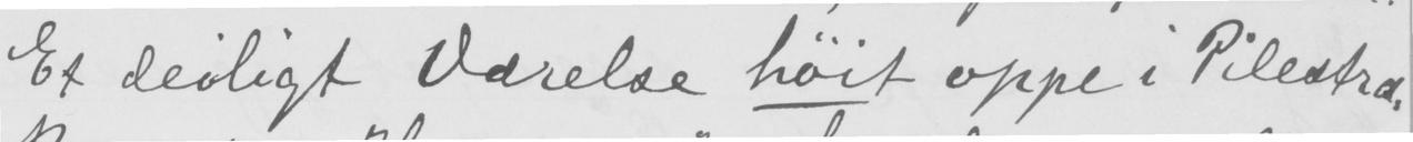Et deiligt Værelse høit oppe i Pilestræ.
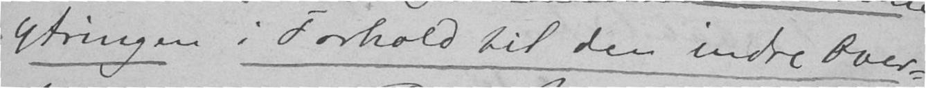ytringen i Forhold til den indre Over-
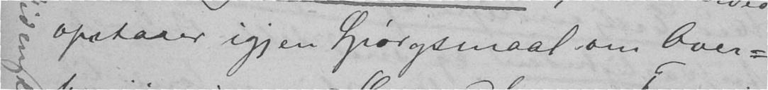opstaaer igjen spørgsmaal om Over-
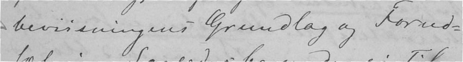beviisningens Grundlag og Forud-
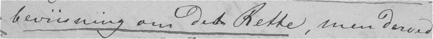beviisning om det Rette, men derved
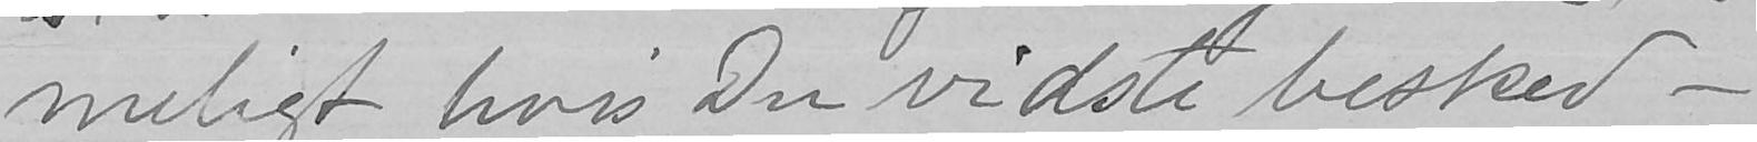meligt hvis Du vidste besked -
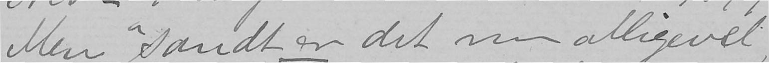Men sandt er det nu alligevel,
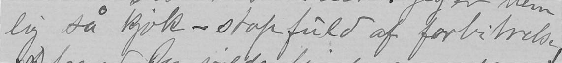lig så kjok-stapfuld af forbitrelse,
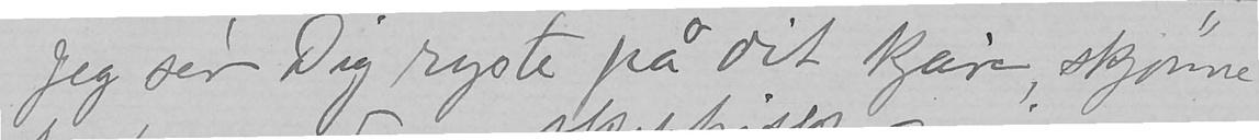Jeg ser Dig ryste på dit kjære, skjønne
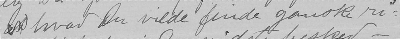at hvad Du vilde finde ganske ri-
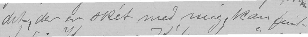det, der er skét med mig, kan finde
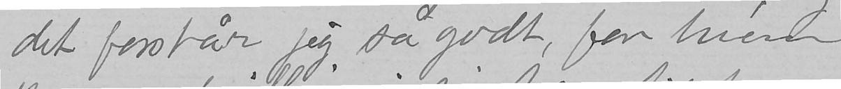det forstår jeg så godt, for hvem
